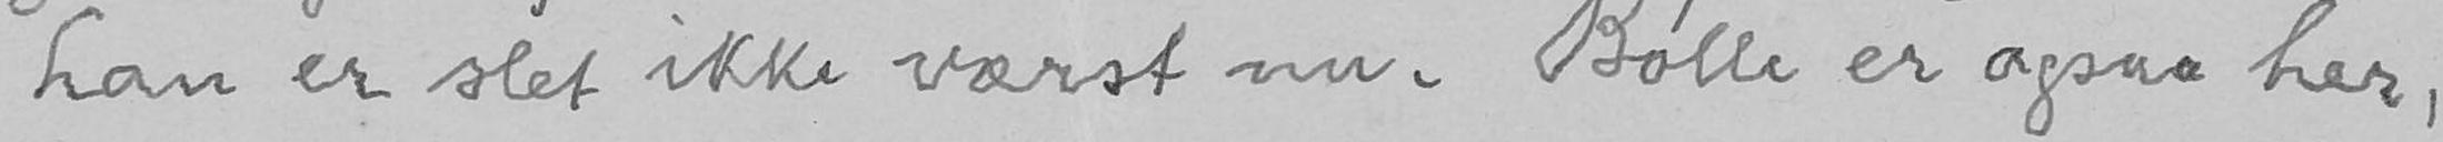han er slet ikke værst nu. Bølle er ogsaa her,
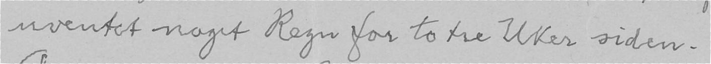uventet noget Regn for to tre Uker siden.
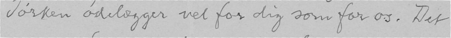Tørken ødelægger vel for dig som for os. Det
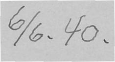6/6. 40.
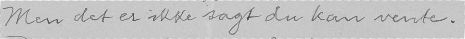Men det er ikke sagt du kan vente.
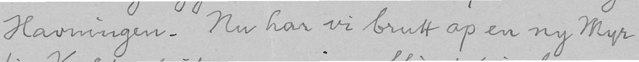Havningen. Nu har vi brutt op en ny Myr
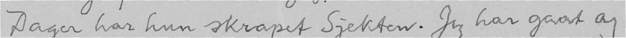Dager har hun skrapet Sjekten. Jeg har gaat og
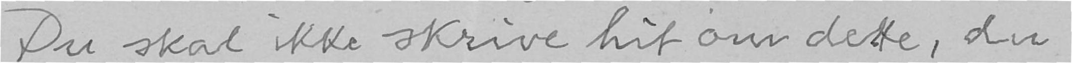Du skal ikke skrive hit om dette, du
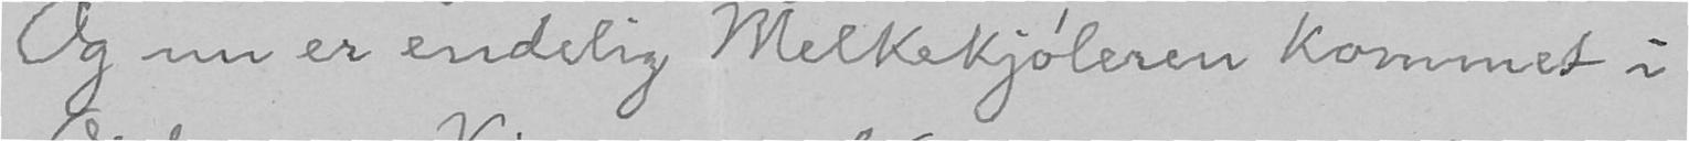Og nu er endelig Melkekjøleren kommet i
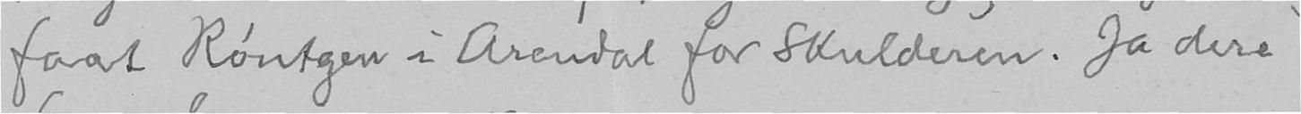faat Røntgen i Arendal for Skulderen. Ja dere
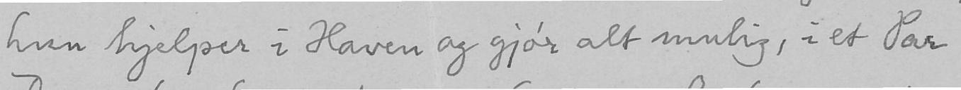hun hjelper i Haven og gjør alt mulig, i et Par
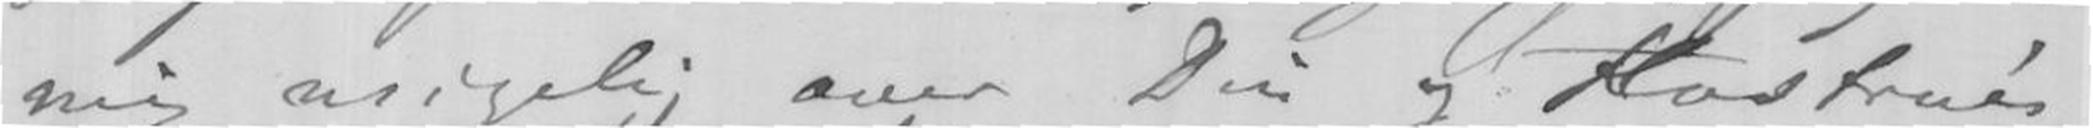mig usigelig over Din og Hustrues
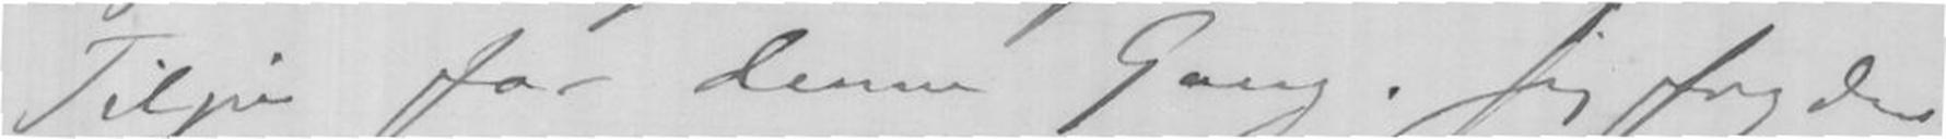Tilgiv for denne Gang. Jeg fryder
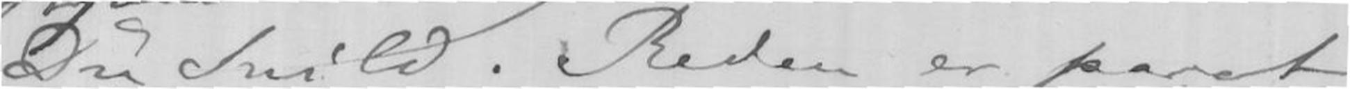Du Snild. Reden er parat
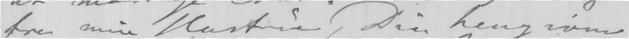fra min Hustru, Din hengivne
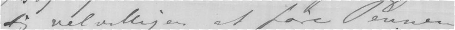sig velvillig at føre Pennen.
